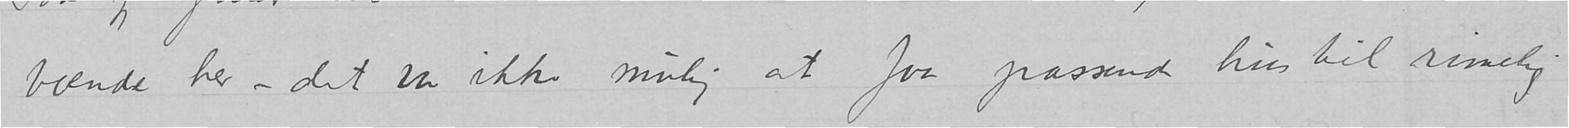boende her – det var ikke mulig at faa passende hus til rimelig
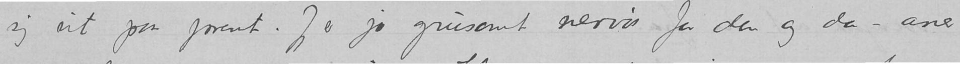sig ut paa prent. Jeg er jo grusomt nervøs for den og da – aner
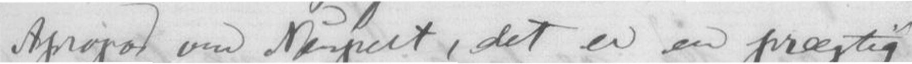Apropos den Neupert, det er en prægtig
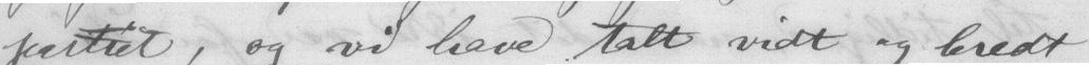partiet, og vi have talt vidt og bredt
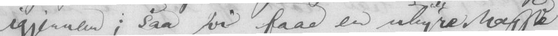igjennem; saa vi faar en uhyre Masse
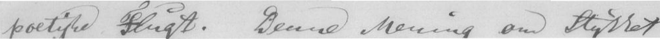poetiske Flugt. Denne Mening om Stykket
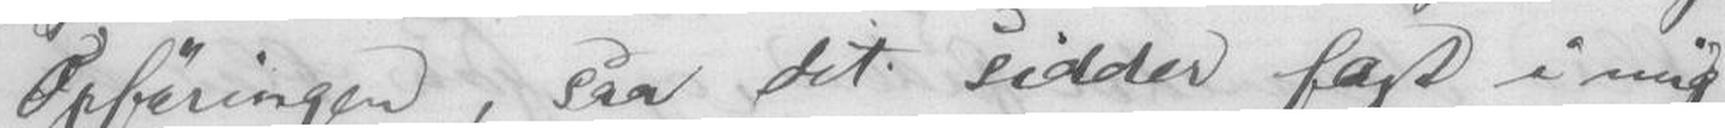Opføringen, saa det sidder fast i mig
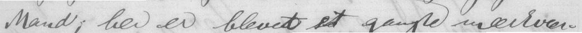Mand; her er blevet et ganske mærkvær-
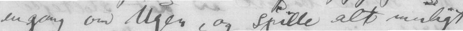engang om Ugen, og spille alt muligt
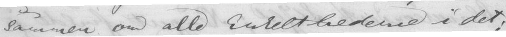sammen om alle Enkelthederne i det;
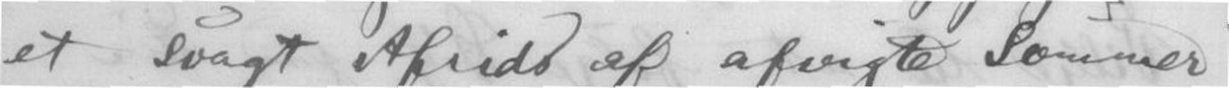et svagt Afrids af afviste Sommer
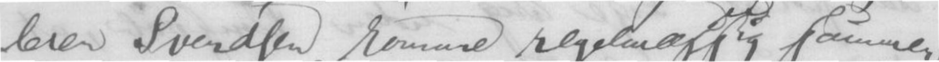leren Svendsen komme regelmæssig sammen
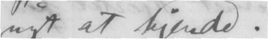nyt at kjende.
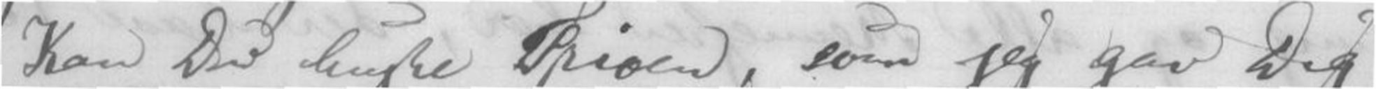Kan Du huske Trioen, som jeg gav Dig
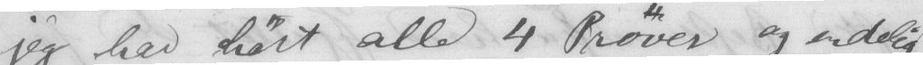jeg har hørt alle 4 Prøver og endelig
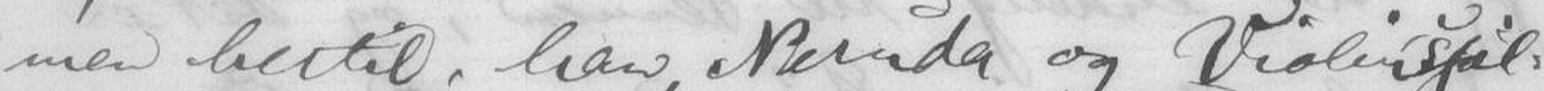men hertil; han, Neruda og Violispil-
